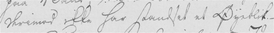derimod ikke har standset et Øyeblik. -
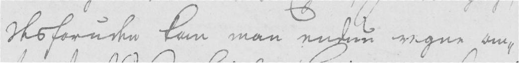desforuden kan man endnu regne om-
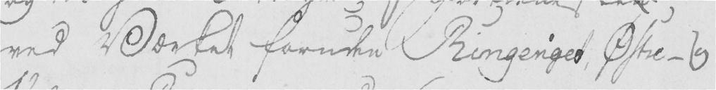ved Værket foruden Ringeriges, Østre- og
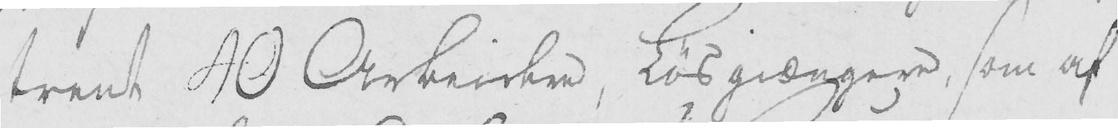trent 40 Arbeidere, Løsgiængere, som af
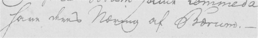have deres Næring af Bærum. -
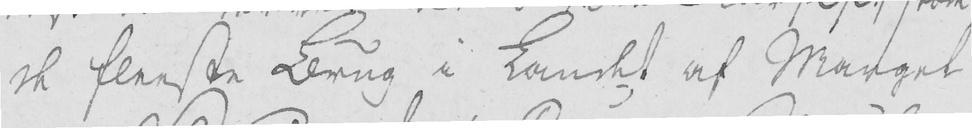de fleeste Brug i Landet af Mangel
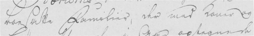boesatte Familier, der med Koner og
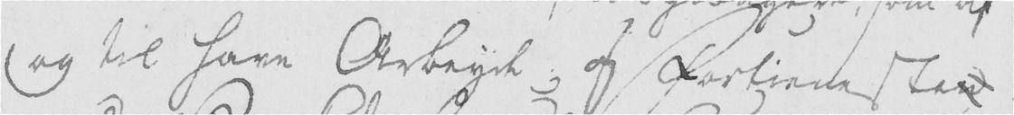og til have Arbeyde; og fortienesten,
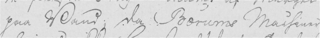paa Vand; da Bærums Machiner
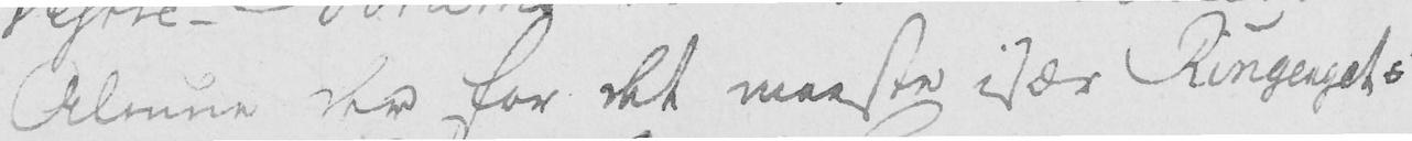Almue der for det meeste især Ringerigets
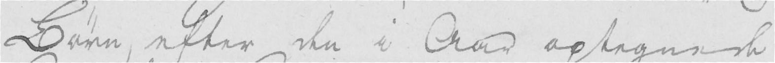Børn, efter den i Aar optegnede
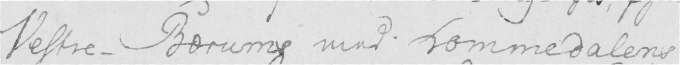Vestre-Bærums med Lommedalens
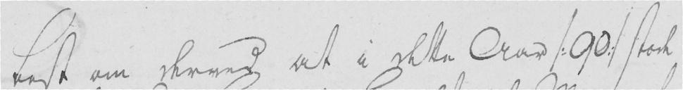best om derved at i dette Aar /: 90 :/ stode
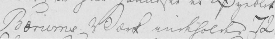Bærums Værk indeholder 72
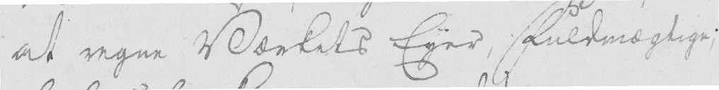at regne Værkets Eyer, Fuldmægtige,
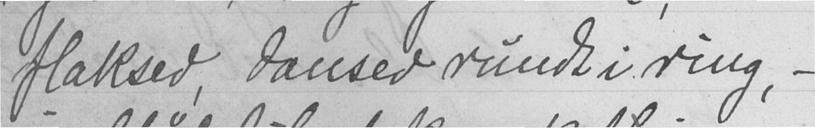flaksed, dansed rundt i ring, -
